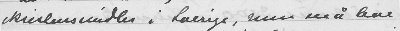kirkens midler i Sverige, men må leve
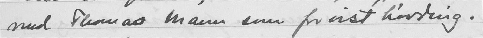med Thomas Mann som forvist høvding.
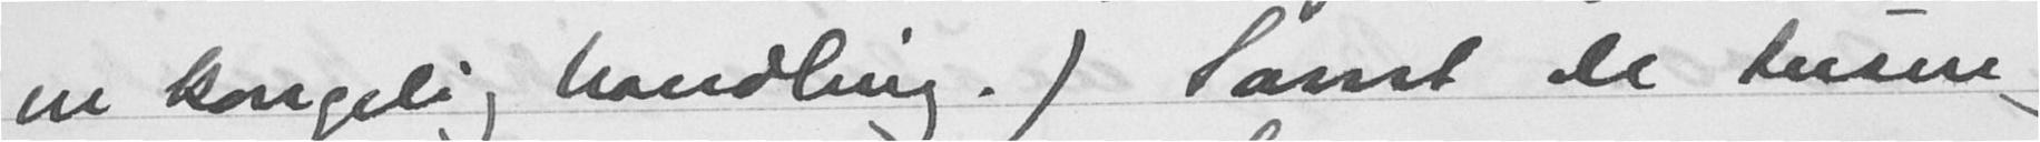en kongelig handling!) Samt de tusen
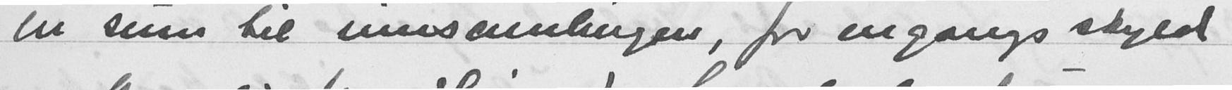en sum til innsamlingen, for engangs skyld,
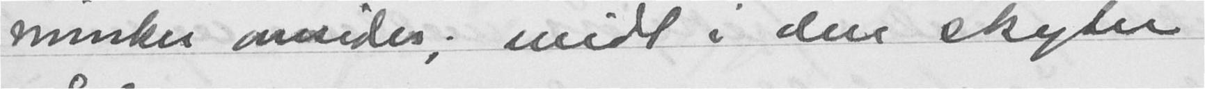minker omsides; midt i den skyter
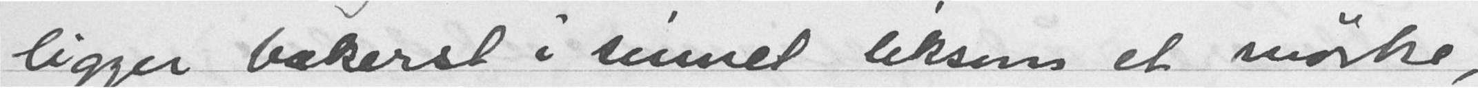ligger bakerst i minnet liksom et mørke,
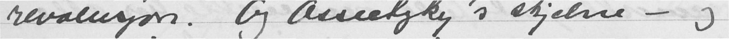revolusjon. Og Ossietzky's skjebne - og
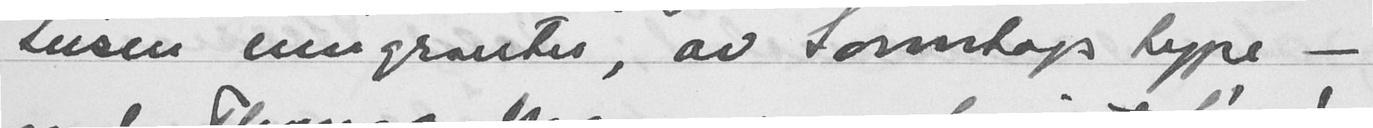tusen emigranter, av Sorentags type -
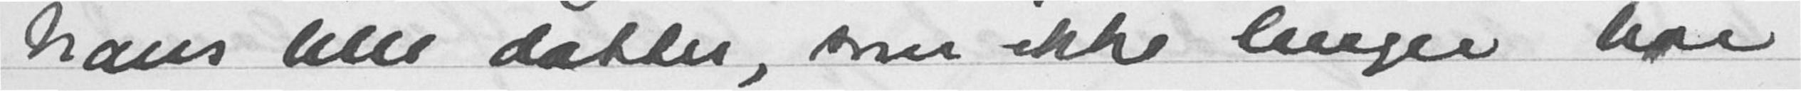hans lille datter, som ikke lenger har
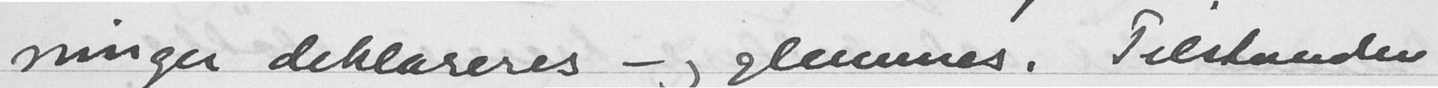ninger deklareres - og glemmes. Tilstander
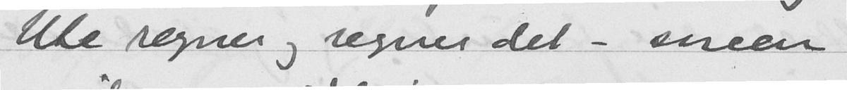Ute regner og regner det - sneen
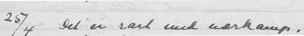25/4 Det er rart med nærkamp,
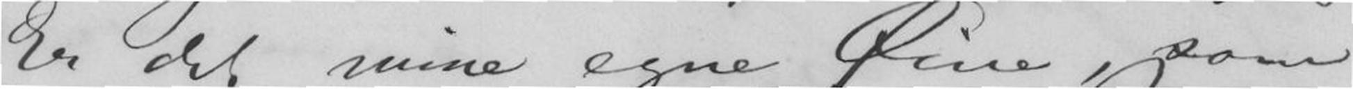Er det mine egne Øine, som
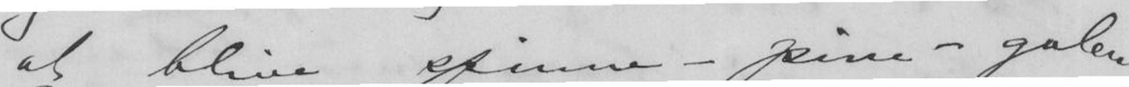at blive spinne- pine- galen.
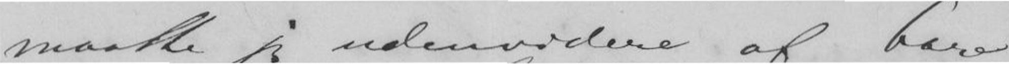maatte jeg udenvidere af bare
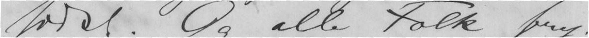sidst. Og alle Folk fry-
